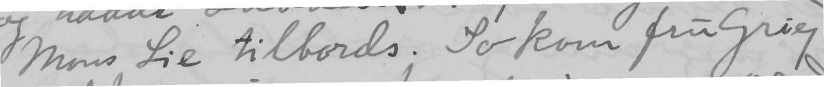Mons Lie tilbords. So kom fru Grieg
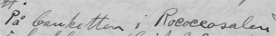På banketten i Roccocosalen
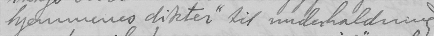hjemmenes dikter" til underhaldning
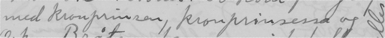med kronprinsen, kronprinsessa og
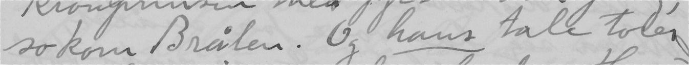so kom Bråten. Og hans tale toler
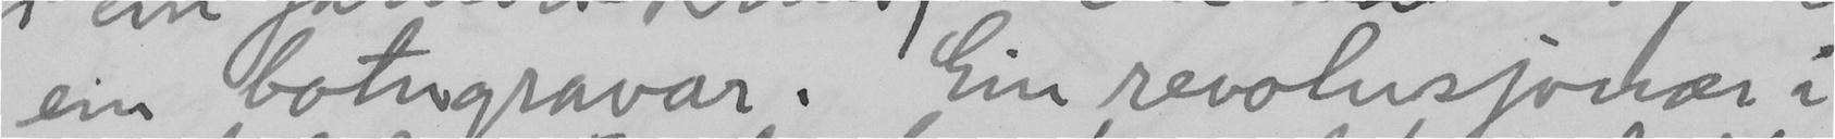ein botngravar. Ein revolusjonær i
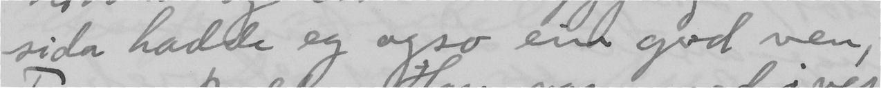sida hadde eg ogso ein god ven,
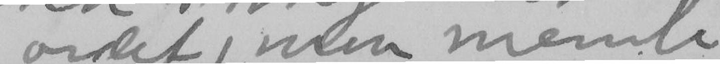ordet, men mente nok slik. Han talla elles for låkt, han som dei andre.
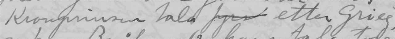Kronprinsen tala  etter Grieg,
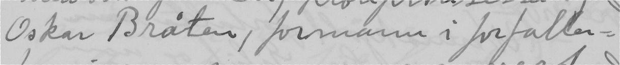Oskar Bråten, formann i fortatter-
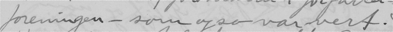foreningen – som ogso var vert.
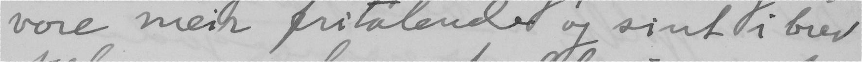vore meir fritalende og sint i brev
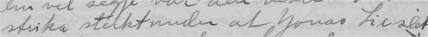strika sterkt under at Jonas Lie slet
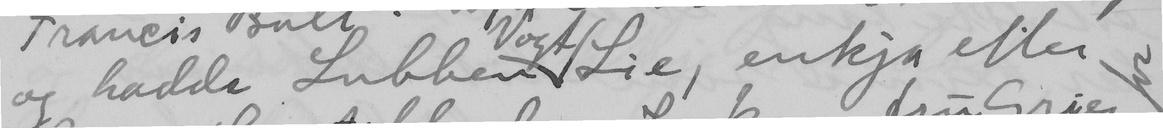og hadde Lubben Vogt Lie, enkja etter
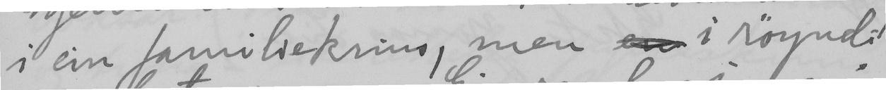i ein familiekrins, men  i röyndi
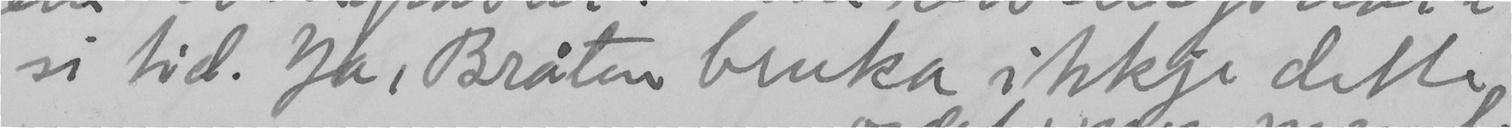si tid. Ja, Bråten bruka ikkje dette
